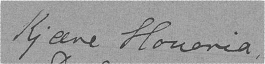Kjære Honoria!
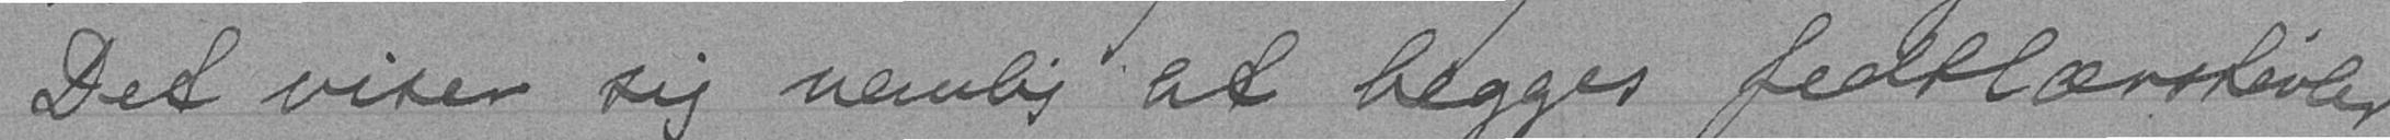Det viser sig nemlig at begges feltlærstøvler
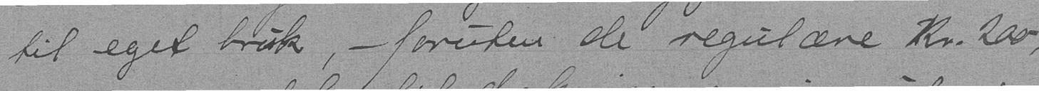til eget bruk, - foruten de regulære Kr. 200,
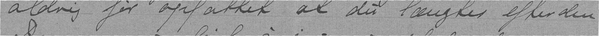aldrig før opfattet at du længter efter den.
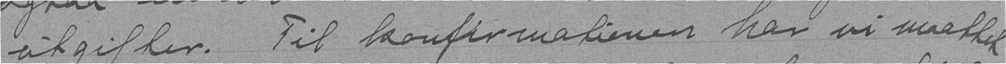udgifter. Til konfirmationen har vi maattet
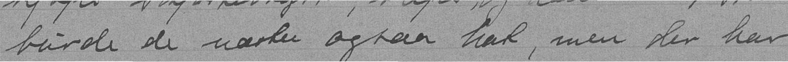burde de næsten ogsaa hat, men der har
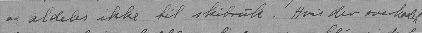og aldeles ikke til skibruk. Hvis der overhodet
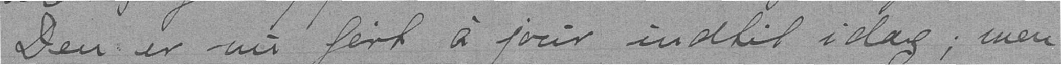Den er nu ført à jour indtil i dag; men
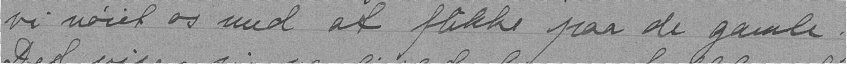vi nøiet os med at flikke paa de gamle.
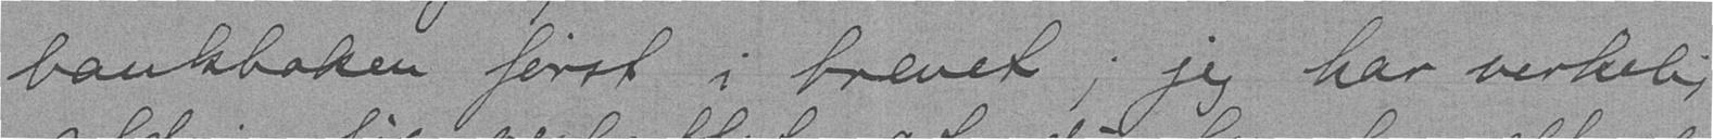bankboken først i brevet; jeg har verkelig
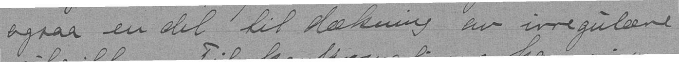ogsaa en del til dækning av irregulære
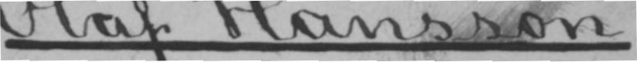Olaf Hansson,
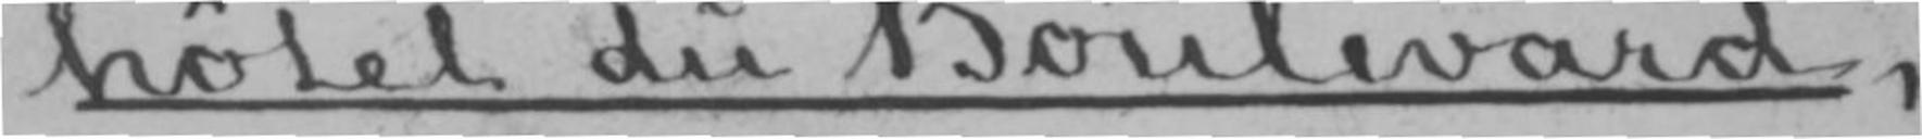hôtel du Boulevard,
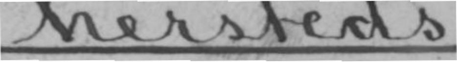hersteds.
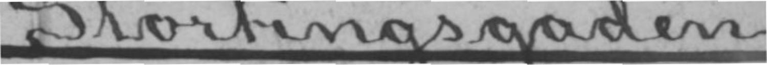Stortingsgaden,
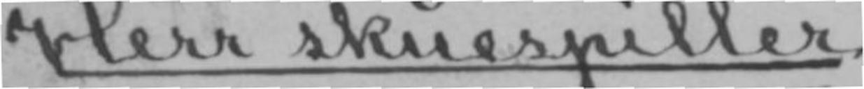Herr skuespiller
